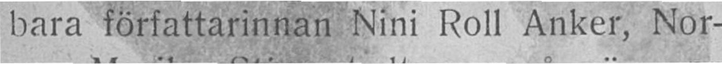bara författarinnan Nini Roll Anker, Nor-
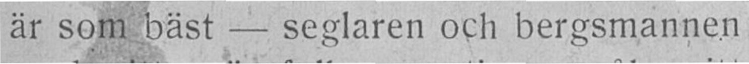är som bäst - seglaren och bergsmannen
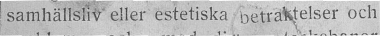samhällsliv eller estetiska betraktelser och
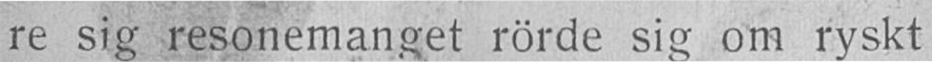re sig resonemanget rörde sig om ryskt
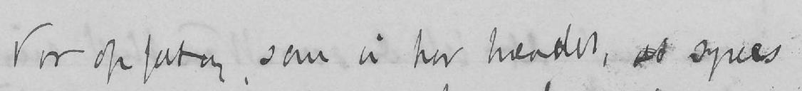Vor opfatning, som vi har hævdet, at synes
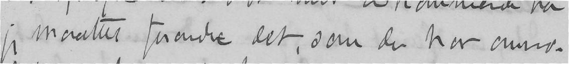jeg maattet forandre det, som den har anmo-
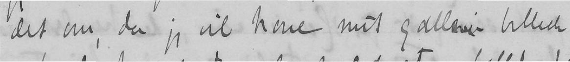det om, da jeg vil have mit galleri billede
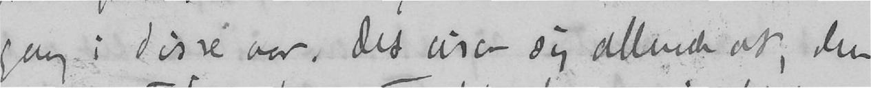gang i disse aar. Det viser sig allerede at, den
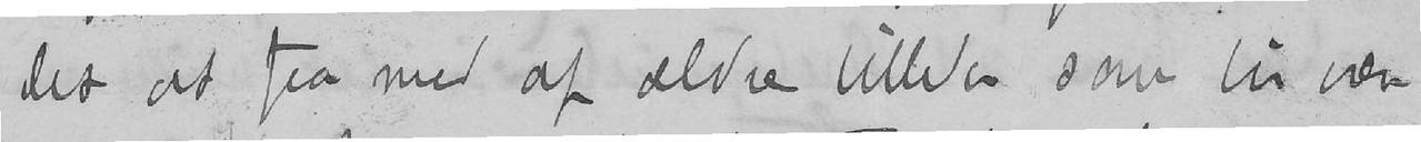det at faa med af ældre billeder som bør være
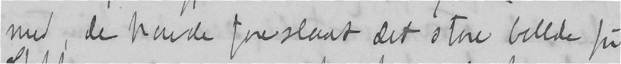med, de havde foreslaat det store billede fra
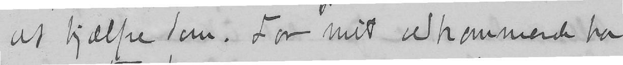at hjælpe dem. For mit vedkommende har
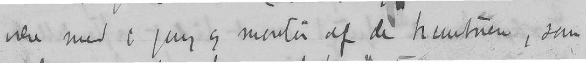være med i jury og montør af de kunstnere, som
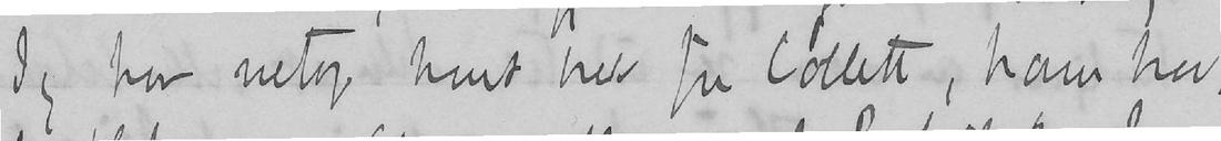Jeg har netop havt her fru Collett, ham har
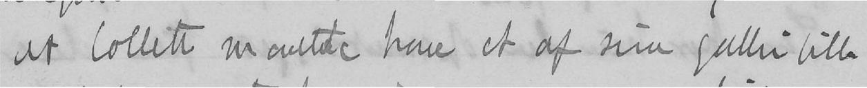at Collett maatte have et af sine galleri bille-
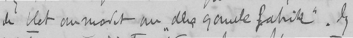de blot anmodet om «den gamle fabrik». Jeg
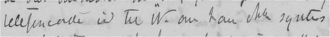telefonerede ud til W. om han ikke syntes
